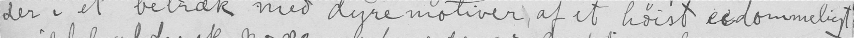der i et betræk med dyremotiver, af et høist udommeligt
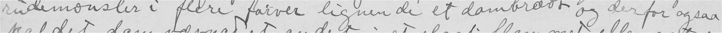rudemønster i flere farver lighende ef dambrædt og derfor ogsaa
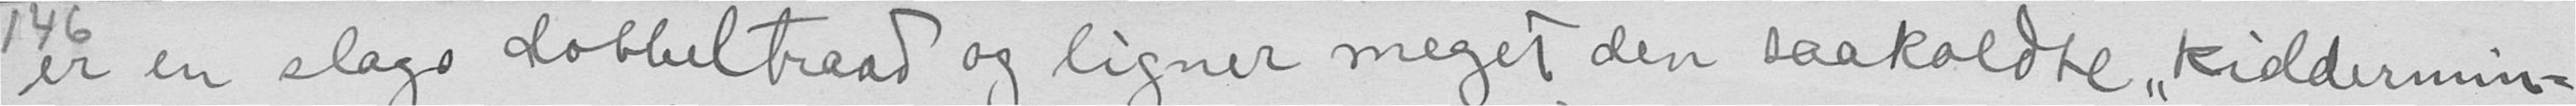er en slags dobbeltraad og ligner meget den saakaldte "kiddermin-
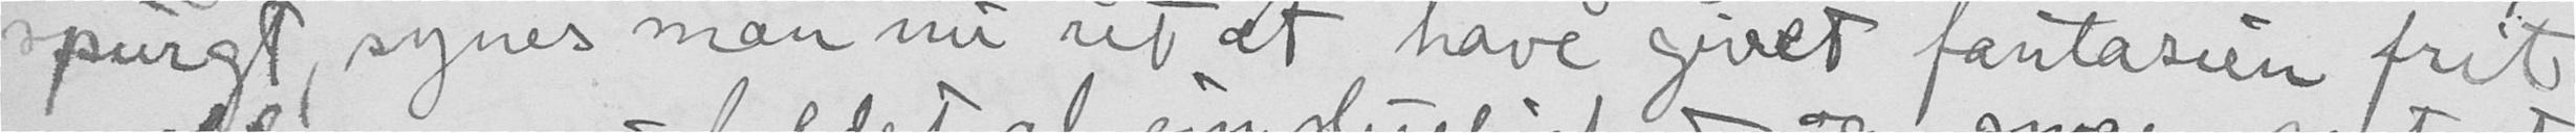spurgt synes man nu ut at have givet fantasien frit
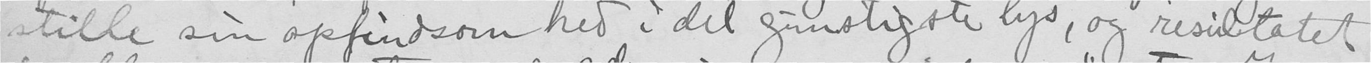stille sin opfindsomhed i det gunstigste lys, og resultatet
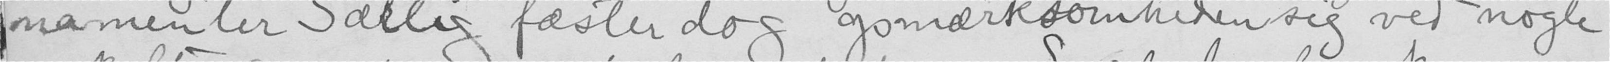namenter Særlig fæster dog opmærksomheden sig vet nogle
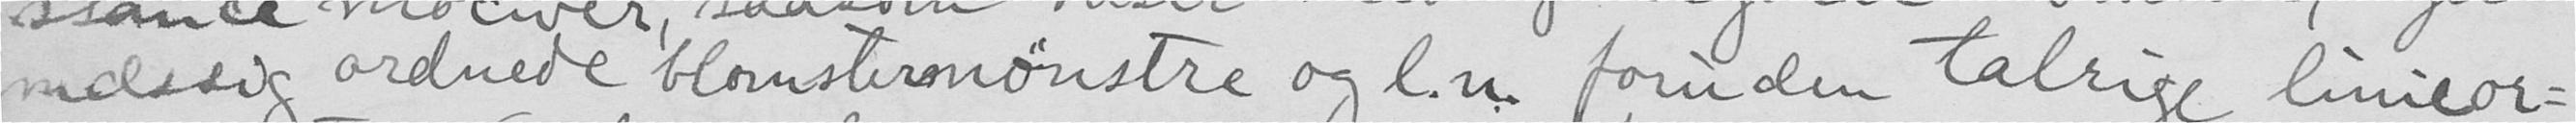mæssig ordnede blomstermønstre og en foruden talrige linieor-
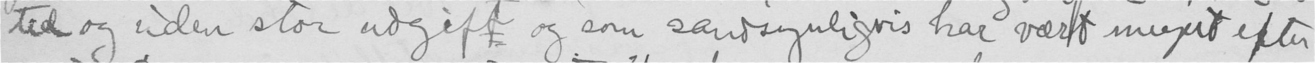tid og uden stor udgift og som sandsynligvis har vært meget efter
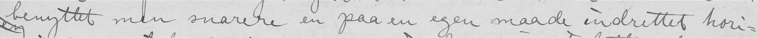benyttet men snarere en paa en egen maade indrettet hori-
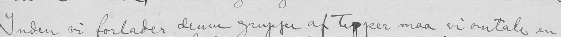Inden vi forlader denne gruppe af tepper maa vi omtale en
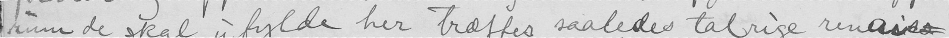rum de skal ufylde her træffes saaledes talrige renais-
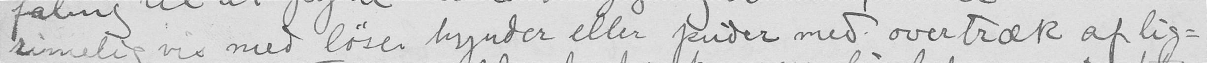rimeligvid med løser hynder eller puder med overtræk af lig-
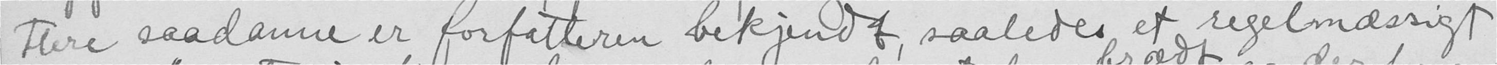Flere saadanne er forfatteren bekjendt saaledes et regelmæssigt
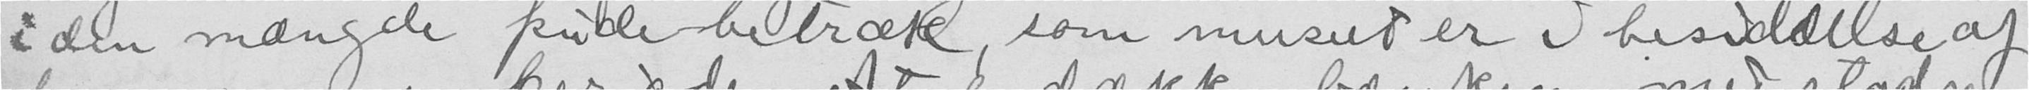i den mængde pude-betræk, som museet er i besiddelse af
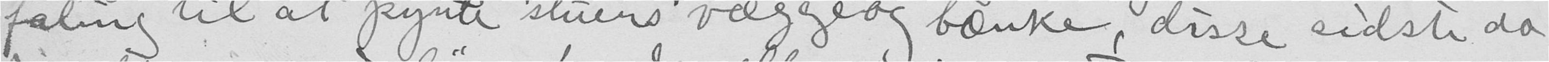faling til at pynte stuens vegge og bænke, disse sidste da
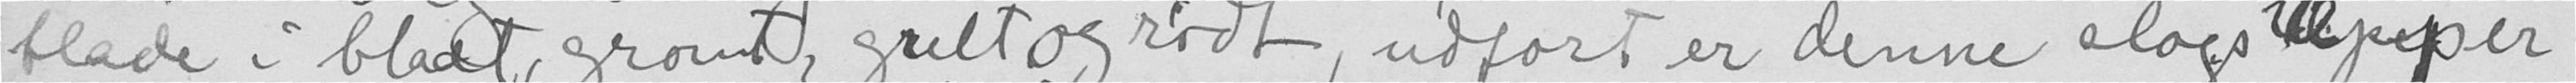blade i blaat, grønt, gult og rødt, udført er denne slags tæpper
